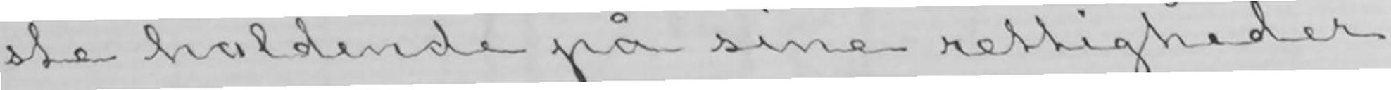ste holdende på sine rettigheder
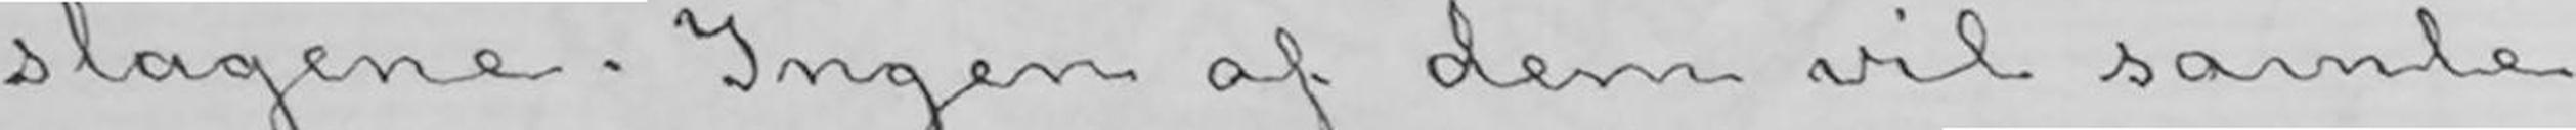slagene. Ingen af dem vil samle
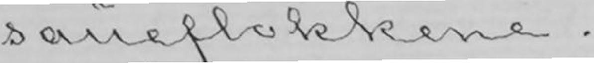saueflokkene.
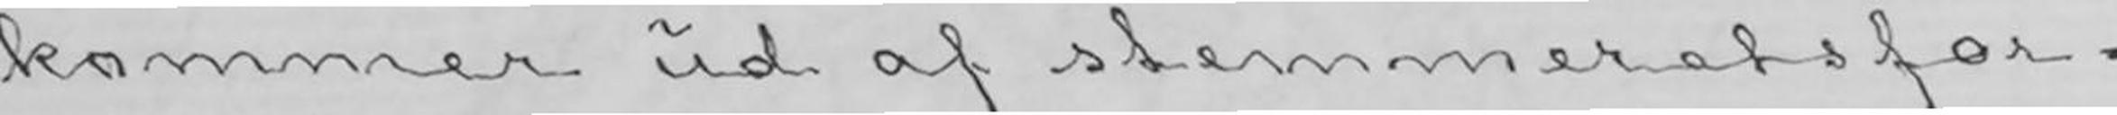kommer ud af stemmeretsfor-
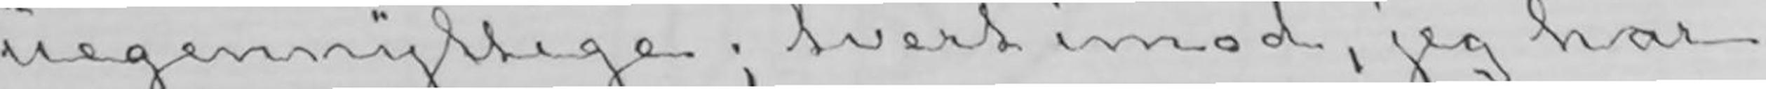uegennyttige; tvert imod, jeg har
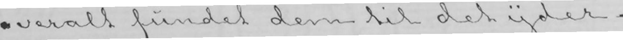overalt fundet dem til det yder-
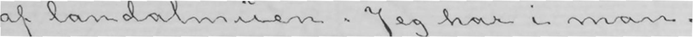af landalmuen. Jeg har i man-
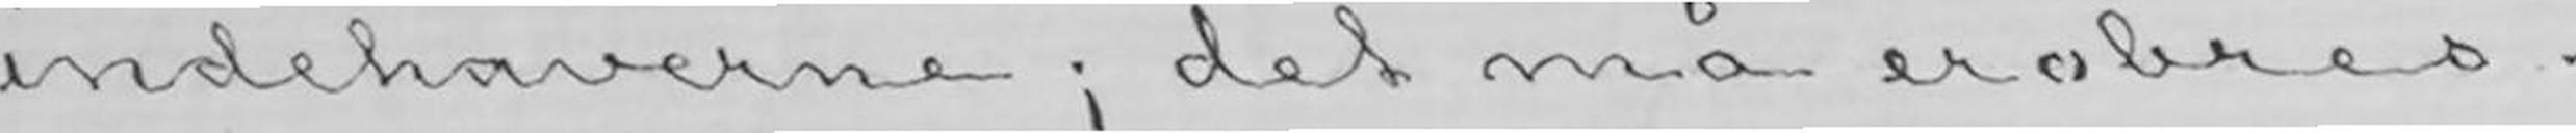indehaverne; det må erobres.
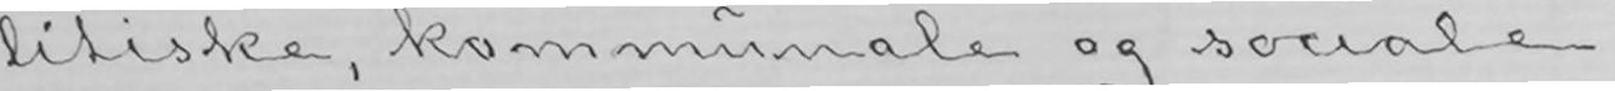litiske, kommunale og sociale
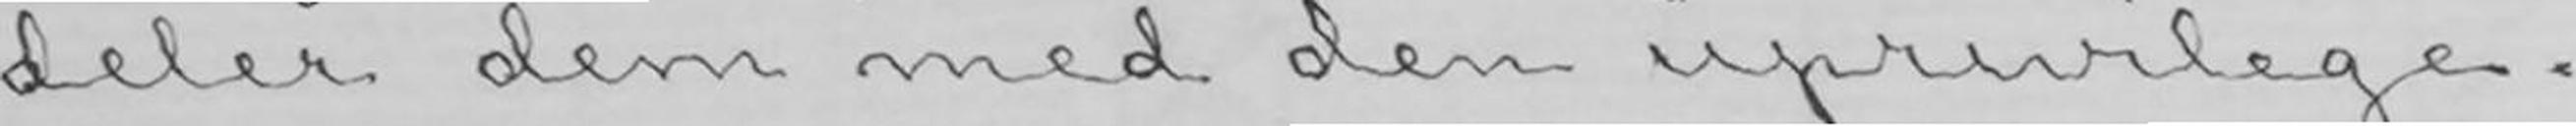deler dem med den uprivilege-
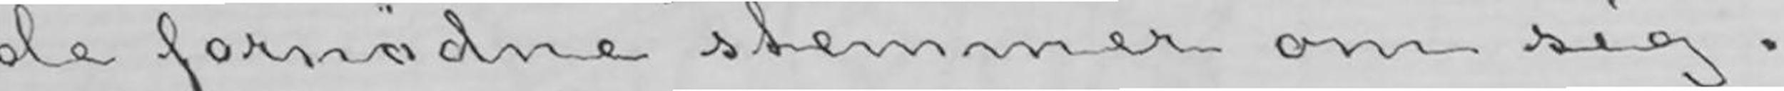de fornødne stemmer om sig.
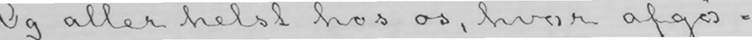Og aller helst hos os, hvor afgø-
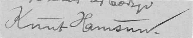Knut Hamsun.
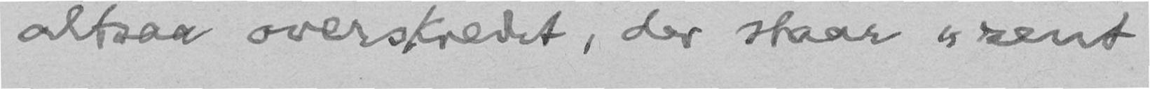altsaa overskredet, der staar "rent
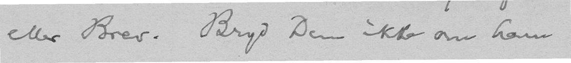eller Brev. Bryd Dem ikke om ham
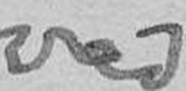ved
